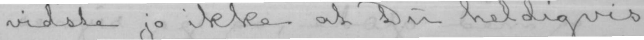vidste jo ikke at Du heldigvis
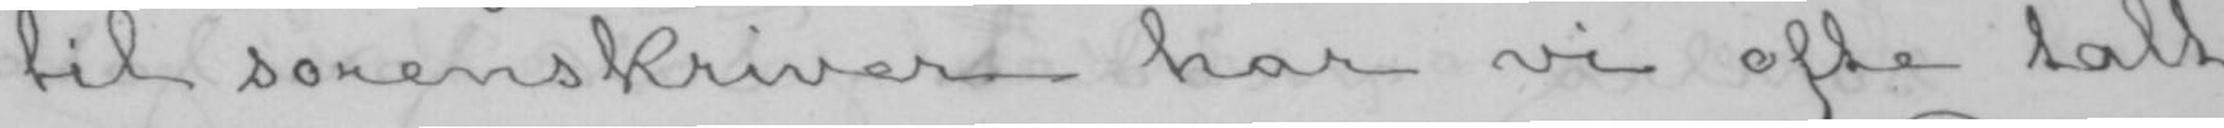til sorenskriver har vi ofte talt
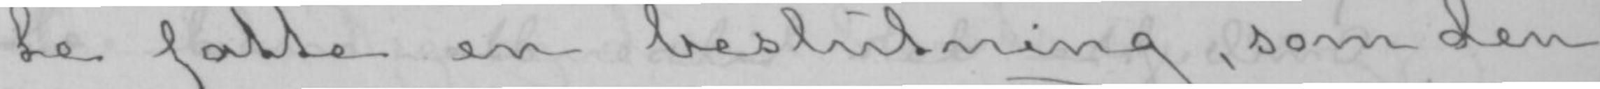te fatte en beslutning, som den
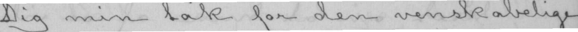Dig min tak for den venskabelige
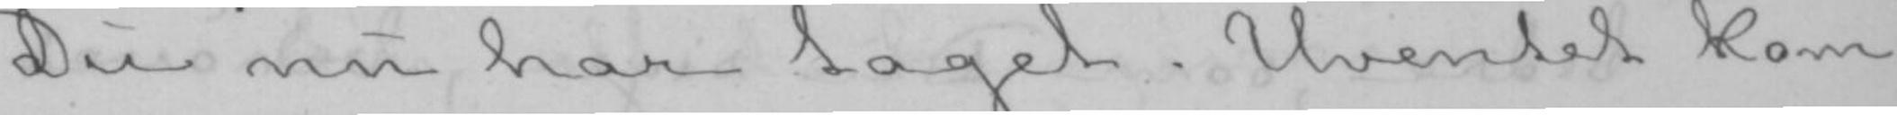Du nu har taget. Uventet kom
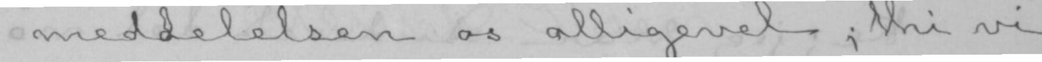meddelelsen os alligevel; thi vi
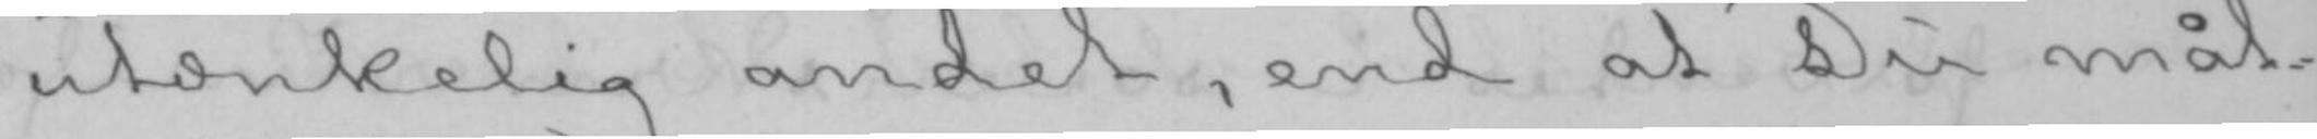utænkelig andet, end at Du måt-
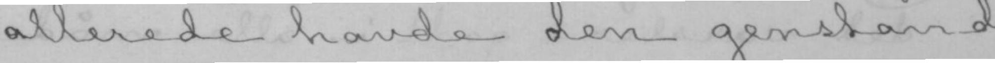allerede havde den genstand
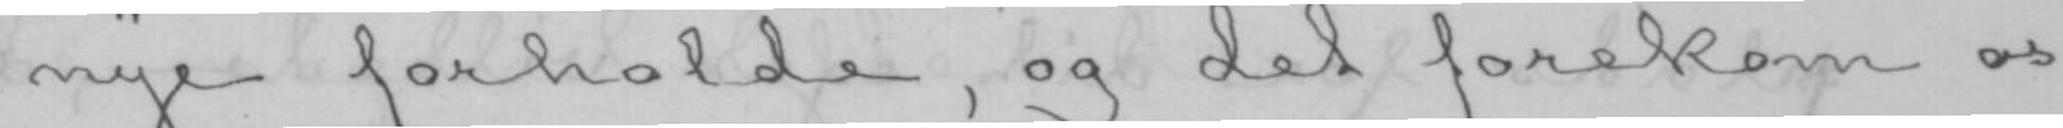nye forholde, og det forekom os
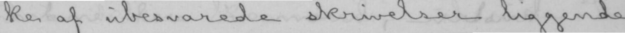ke af ubesvarede skrivelser liggende
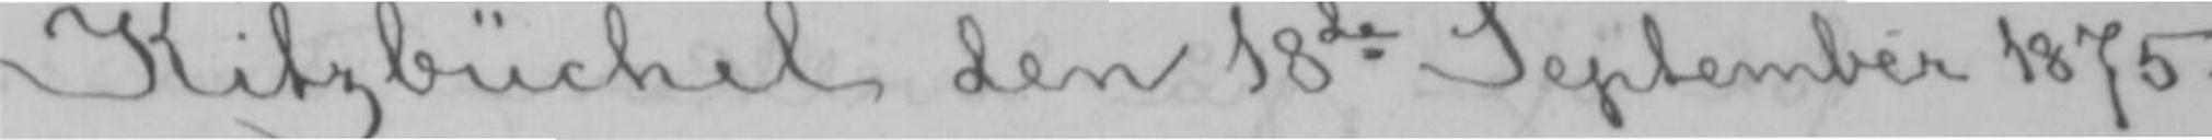Kitzbüchel den 18de September 1875.
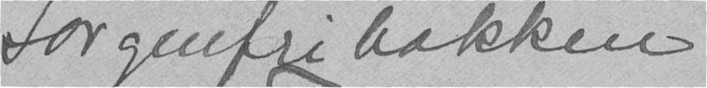Sorgenfribakken
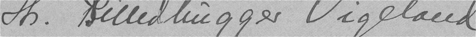Hr. Billedhugger Vigeland
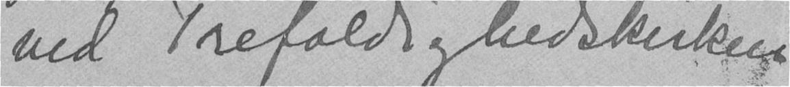ved Trefoldighedskirken
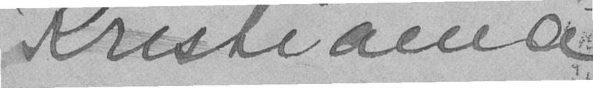Kristiania
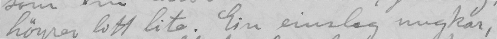höyrer litt lite. Ein einsleg ungkar,
